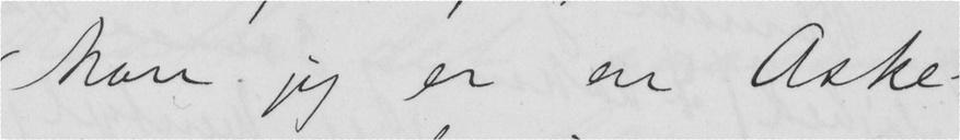Men jeg er en Aske
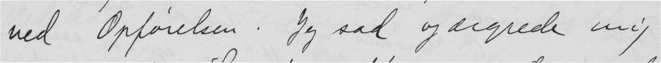ved Opførelsen. Jeg sad og ærgrede mig
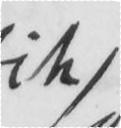fik
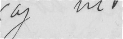og vi
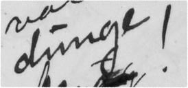dunge!
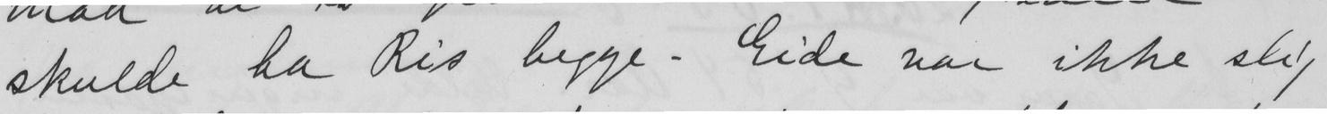skulde ha Ris begge. Eide var ikke slig
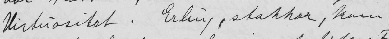Virtuøsitet. Erling, stakkar, kom
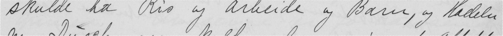skulde ha Ris og Arbeide og Barn, og Hadeln
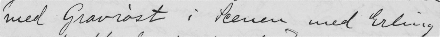med Gravrøst i Scenen med Erling
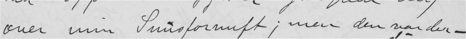over min Snusfornuft; men den var der -
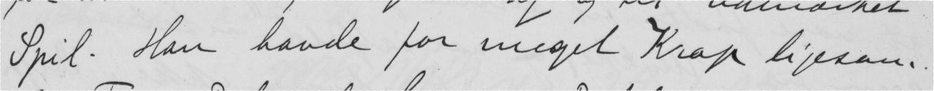Spil. Han havde for meget Krop ligesom.
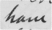ham
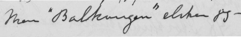Men "Balkongen" elsker jeg -
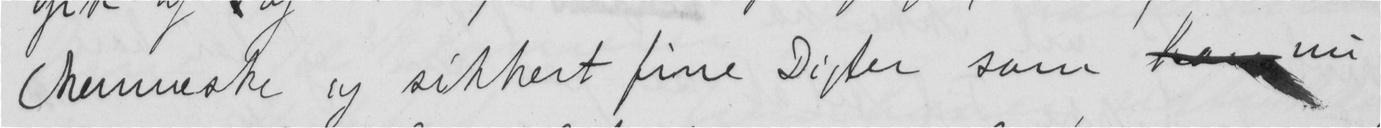Menneske og sikkert fine Digter, som  nu
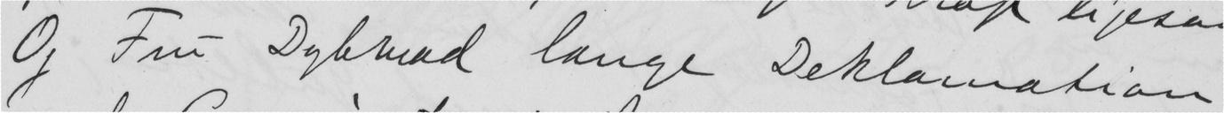Og Fru Dybwad lange Deklamation
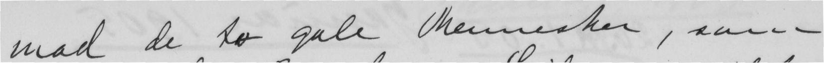mod de to gale Mennesker, som
